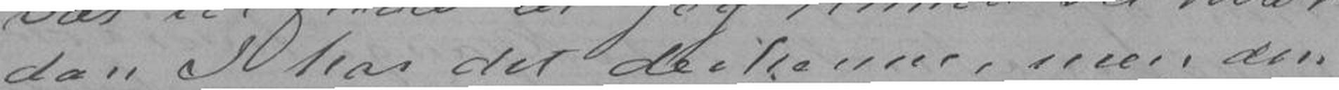dan I har det derhenne, men den
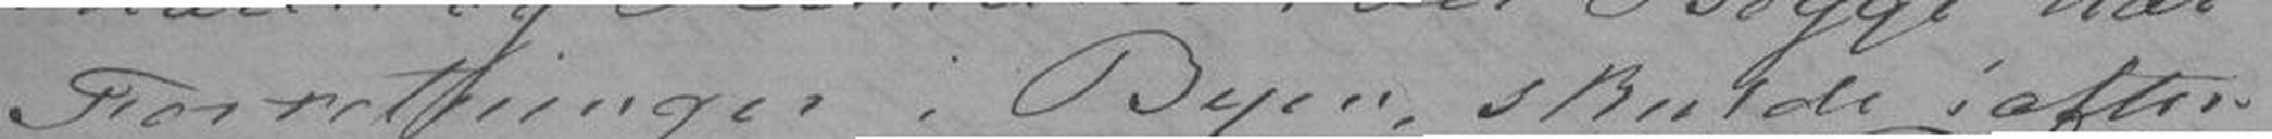Forretninger i Byen, skulde iaften
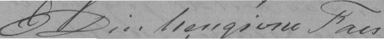Din hengivne Faer
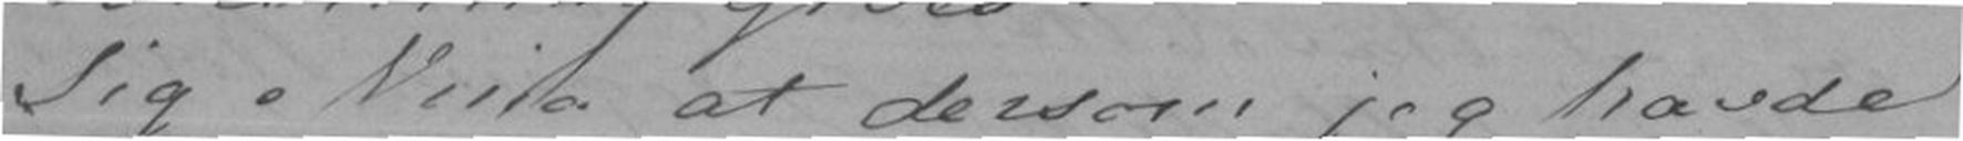Sig Nina at dersom jeg havde
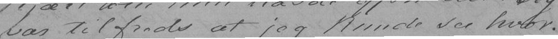var tilfreds at jeg kunde see hvor-
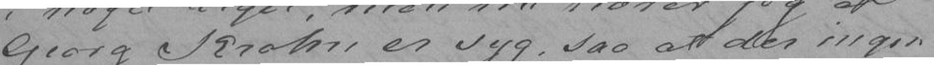Georg Krohn er syg, saa at der ingen
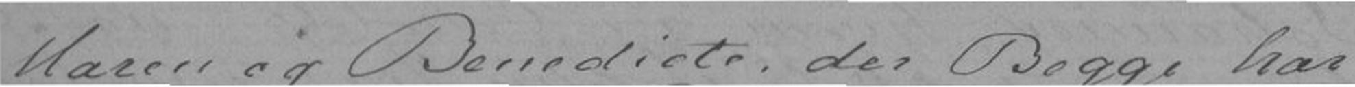Maren og Benedicte, der Begge har
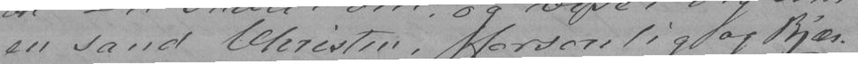en sand Christen, forsonlig og kjær-
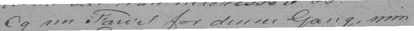Og nu Farvel for denne Gang, min
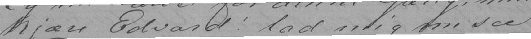kjære Edvard! lad mig nu see
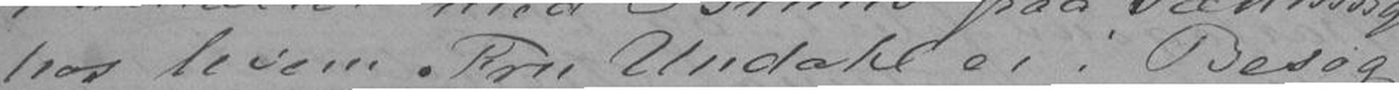hos hvem Fru Undahl er i Besøg
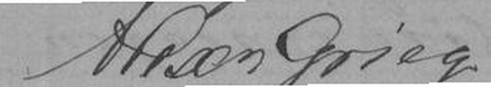Alexr Grieg
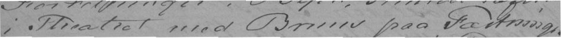i Theatret med Bruns paa Fæstningen
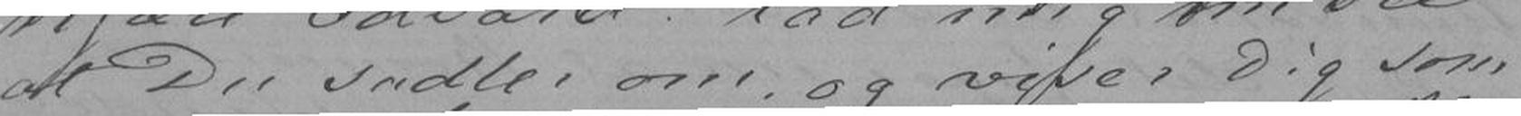at Du sadler om, og viser Dig som
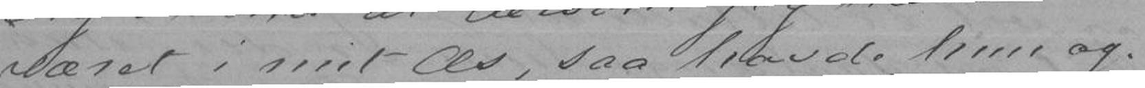været i mit Æs, saa havde hun og-
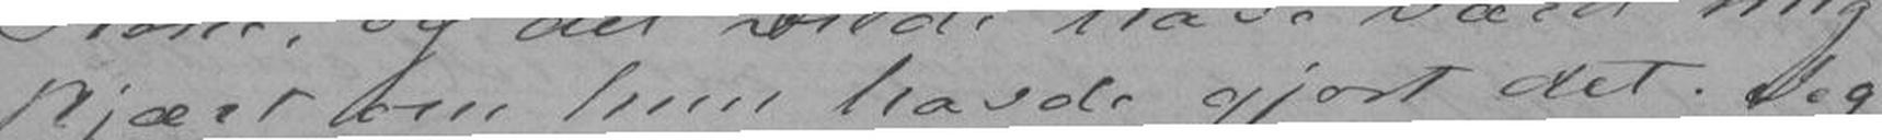kjært om hun havde gjort det. Jeg
